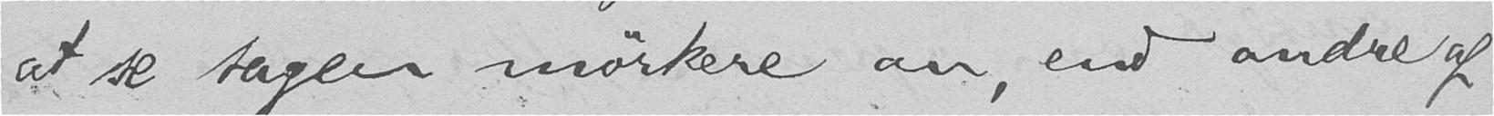at se sagen mørkere an, end andre af
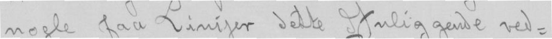nogle faa Linijer dette Anliggende ved-
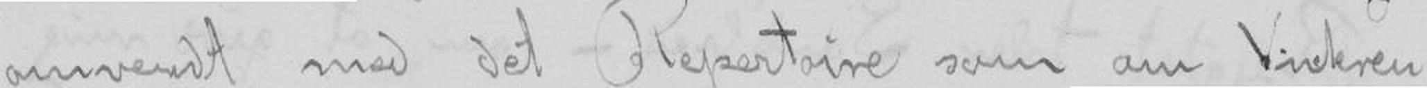omvendt med det Repertoire som om Vinteren
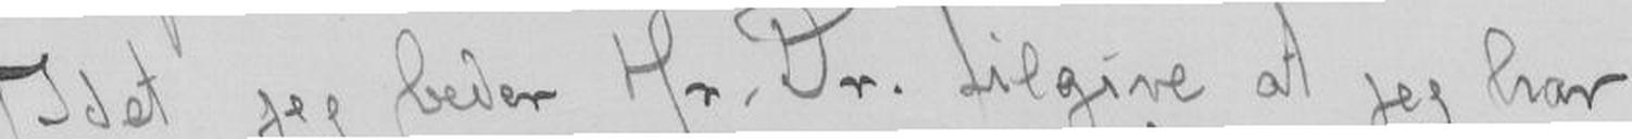Idet jeg beder Hr. Dr. tilgive at jeg har
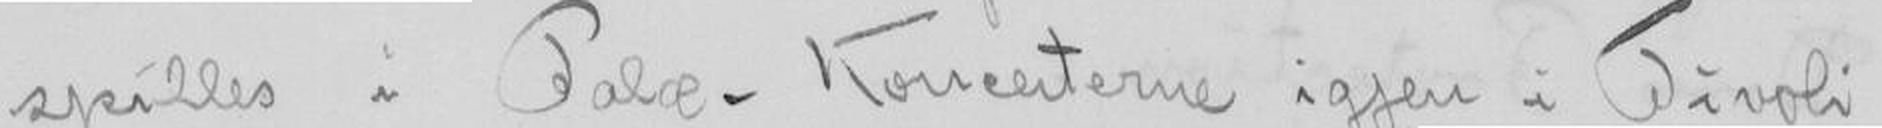spilles i Palæ-Koncerterne igjen i Tivoli
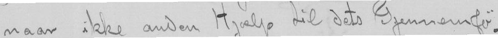naar ikke anden Hjælp til dets Gjennemfø-
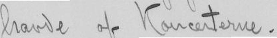havde af Koncerterne.
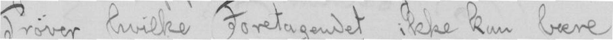Prøver hvilke Foretagendet ikke kan bære
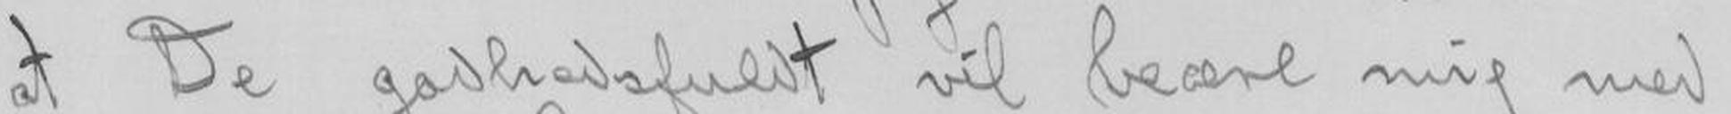at De godhedsfuldt vil beære mig med
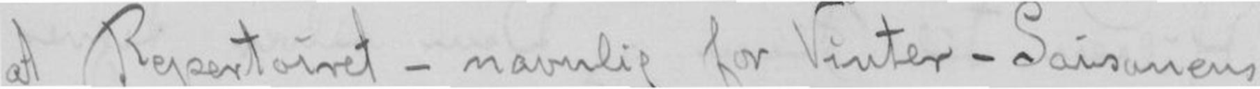at Repertoiret - navnlig for Vinter-Saisonens
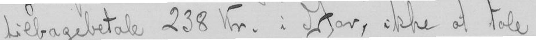tilbagebetale 238 Kr. i Aar, ikke at tale
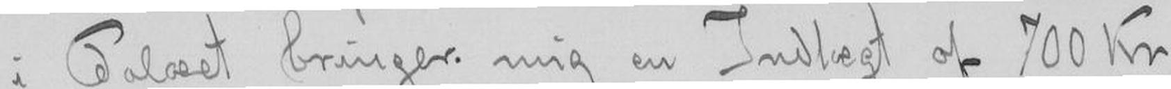i Palæet briger mig en Indtægt af 700 Kr.
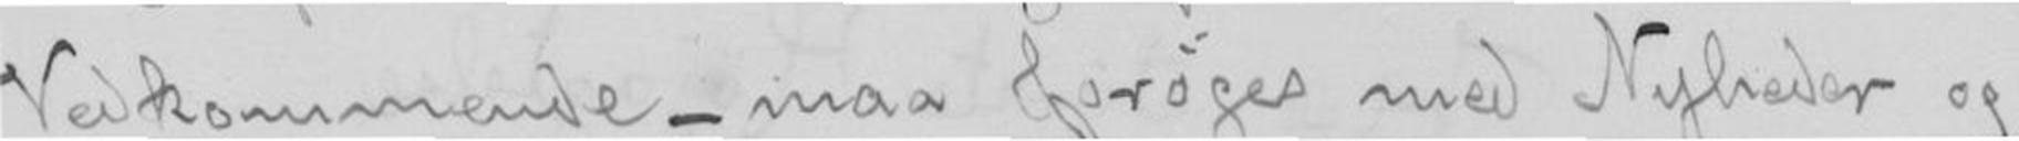Vedkommende - maa forøges med Nyheder og
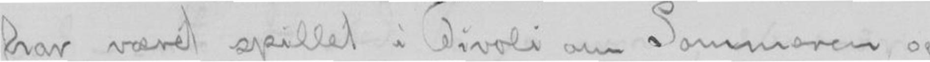har været spillet i Tivoli om Sommeren og
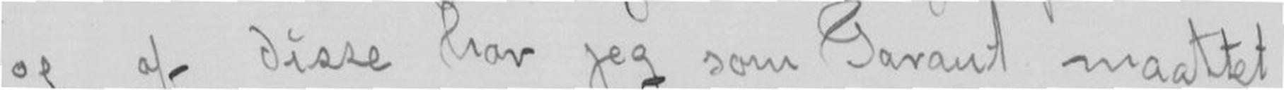og af disse har jeg som Garant maattet
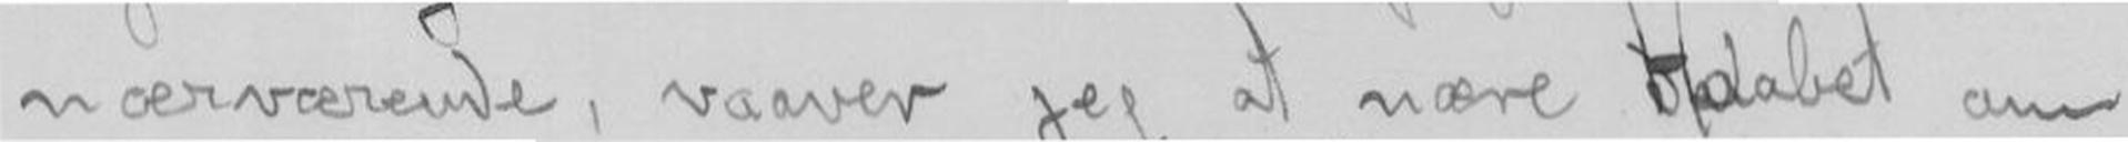nærværende, vaaver jeg nære Haabet om,
